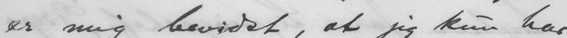er mig bevidst, at jeg kun har
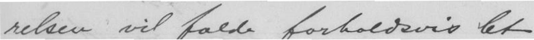relsen vil falde forholdsvis let
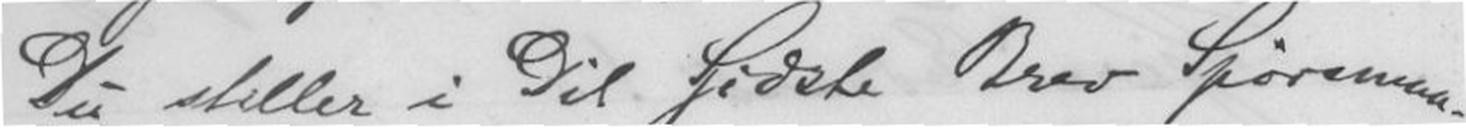Du stiller i Dit Sidste Brev Spørsmaa-
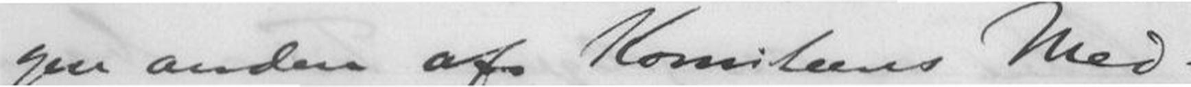gen anden af Komiteens Med-
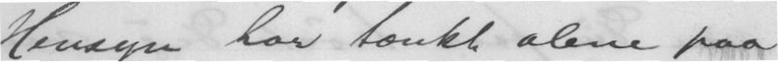Hensyn har tænkt alene paa
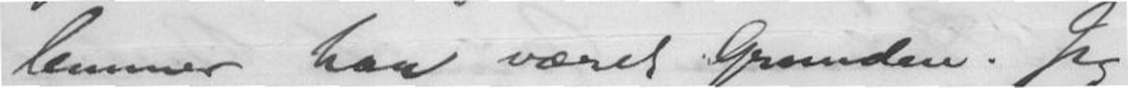lemmer har været Grunden. Jeg
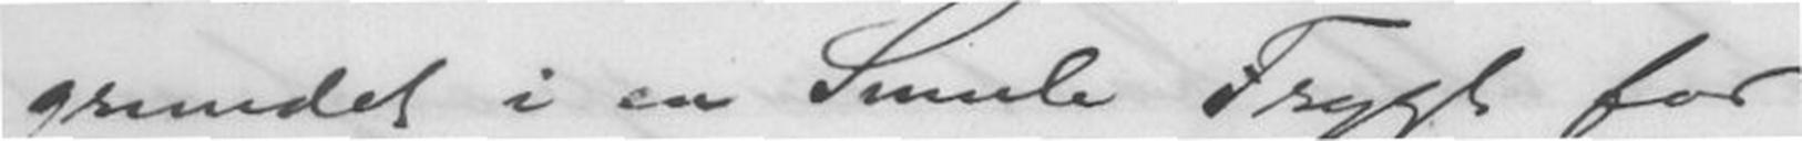grundet i en Smule Frygt for
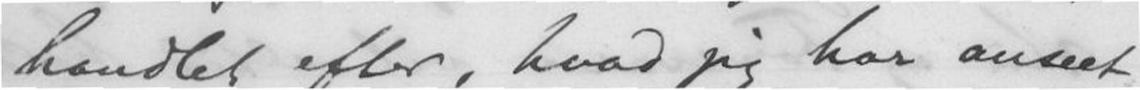handlet efter, hvad jeg har anseet
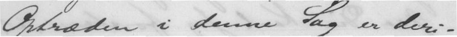Optræden i denne Sag er deri-
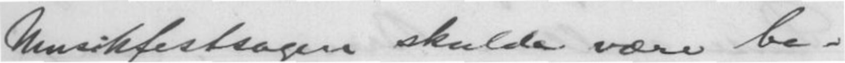Musikfestsagen skulde være be-
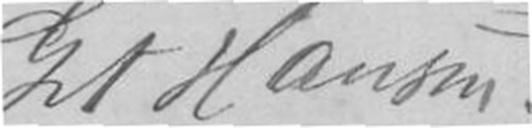G.A. Hansen
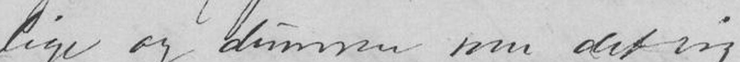lige og dumme som det
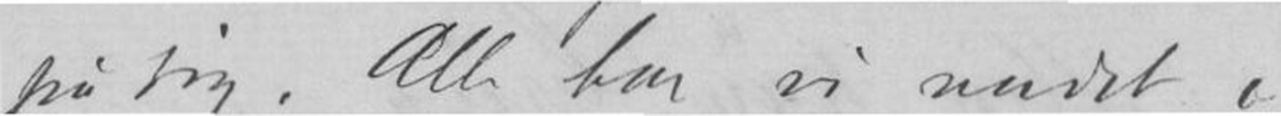på sig. Alle har vi været i
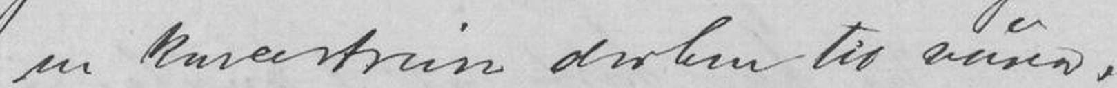en derhen til våren,
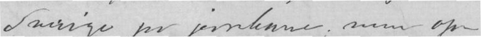Sverige fra jernbane; men op
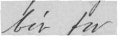bør for
